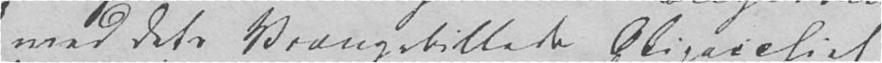med dets Vrængebillede Oligarchiet,
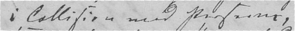i Collision med Perserne.
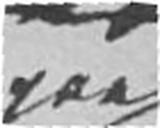paa
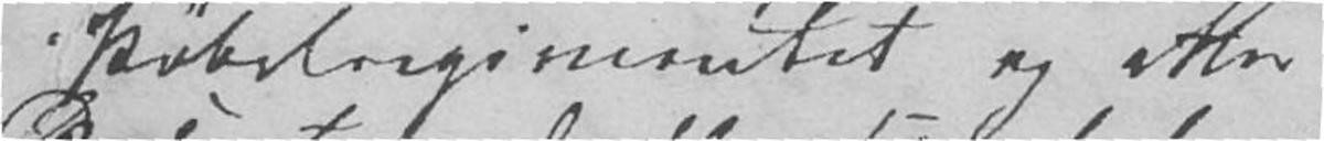Pøbelregimentet og atter
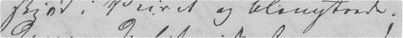skjød i Veiret og blomstrede.
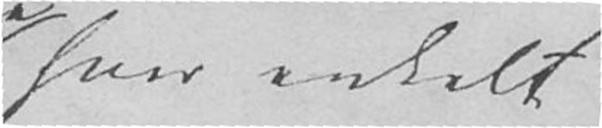hver enkelt
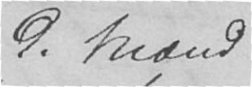de Mænd
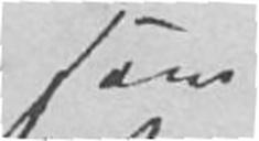som
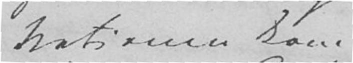Nationen kom
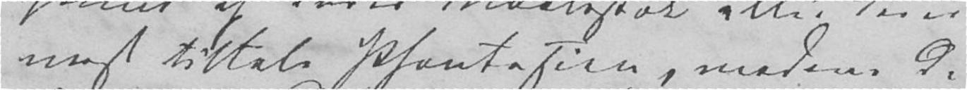mest tiltale Phantasien, medens de
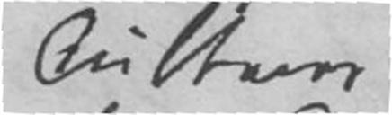Culturer
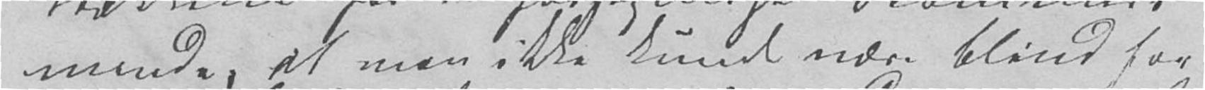mende, at man ikke kunde være blind for
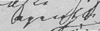Eens
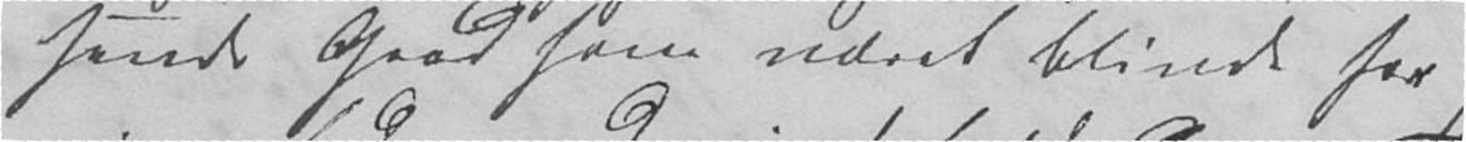sende Grad have været blinde for
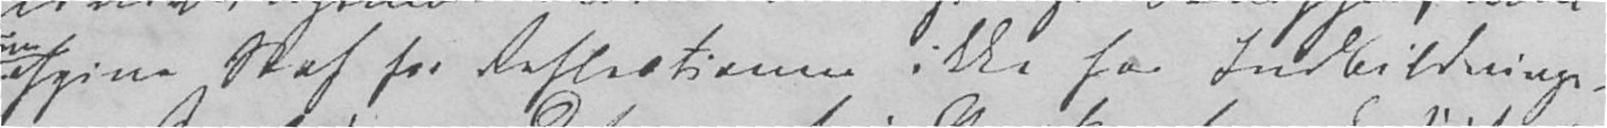afgive Stof for Reflectionen ikke for Indbildnings-
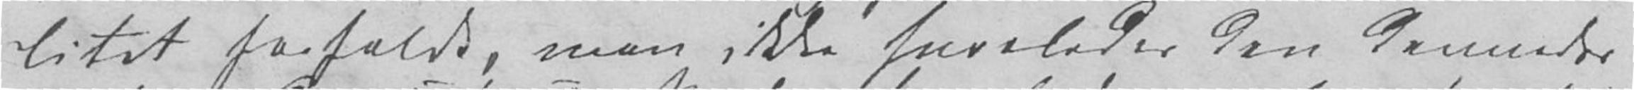litet forfaldt, men ikke hvorledes den dannedes
